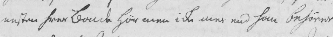nesten hver Bonde Hør men ikke mer end han befører
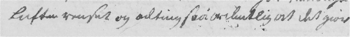Luften renset og alting saa ordentlig at det giør
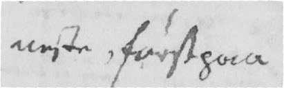neste, først paa
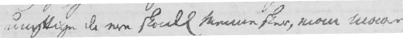unyttige de ere skadl Mennesker, man maae
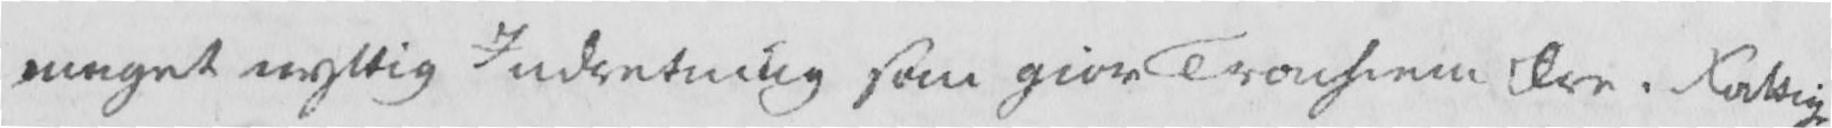meget nyttig Indretning som giør Tronhiem Ære. Fattige
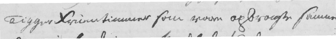Tigger Fruentimmer som vare opbragte samme
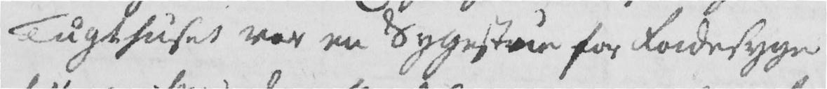Tugthuset var en Sygestue for Radesyge
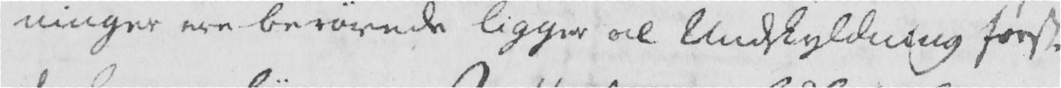ninger ere berøvede ligger al Undskyldning først
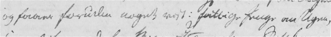og faaer foruden noget vist. fattige Penge om Ugen,
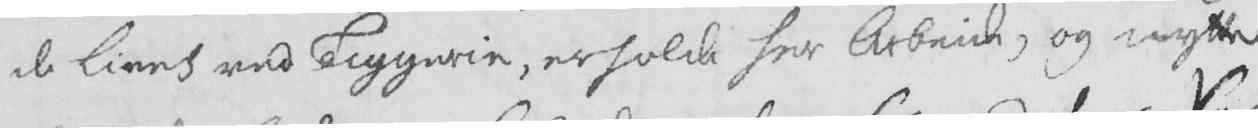de livet ved Tiggerie, erholde her Arbeide, og nytte
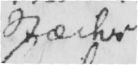Stæder
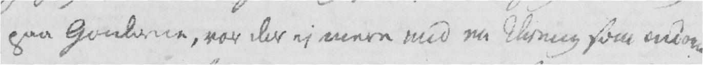paa Gaderne, var der ej mere end en Dreng som incom-
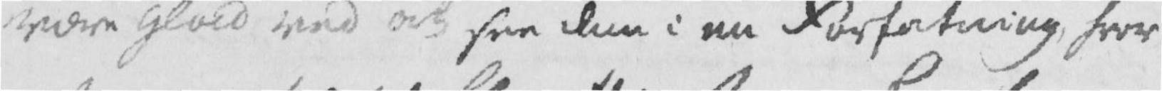være glad ved at see dem i en Forfatning, hvor
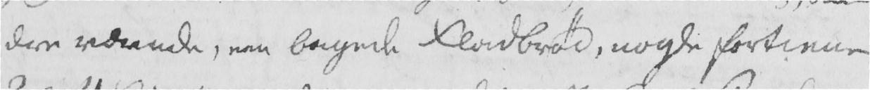dre vævede, een bagede Fladbrød, nogle fortiene
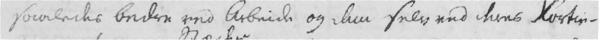saaledes bedre ved Arbeide og dem selv ved deres Fortie-
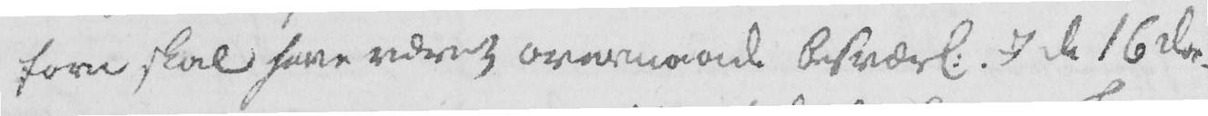forn skal have været overmaade besværl. I de 16 da-
